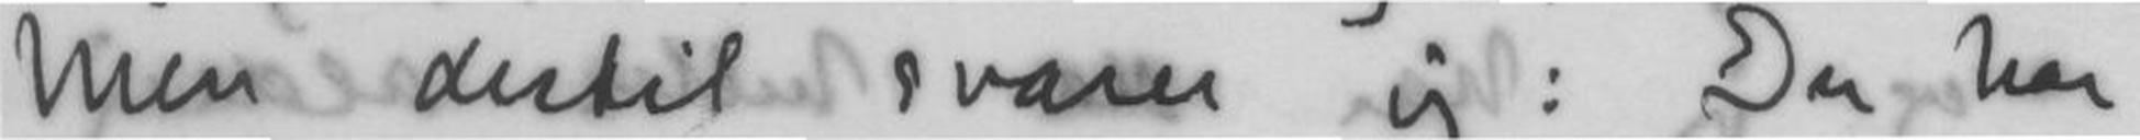Men dertil svarer jeg: Du har
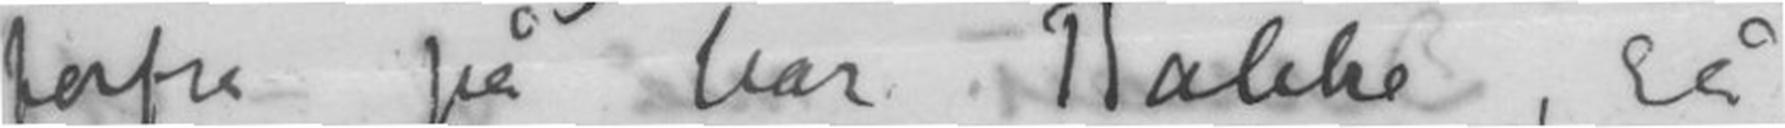forfra på bar Bakke, så
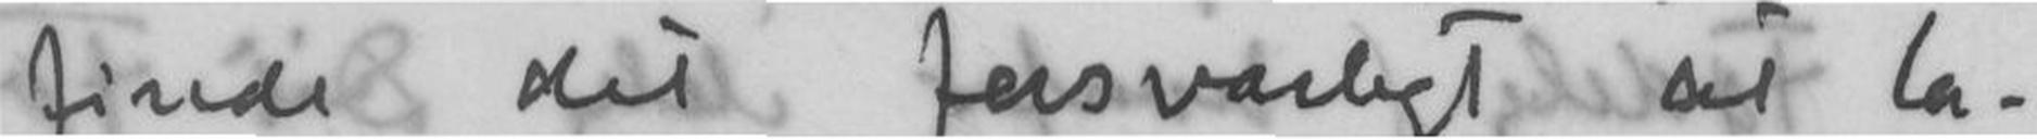finde det forsvarligt at la-
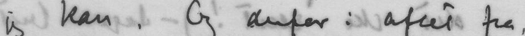jeg kan. Og derfor i afset fra,
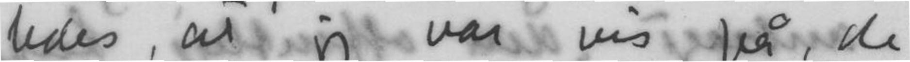ledes, at jeg var vis på, de
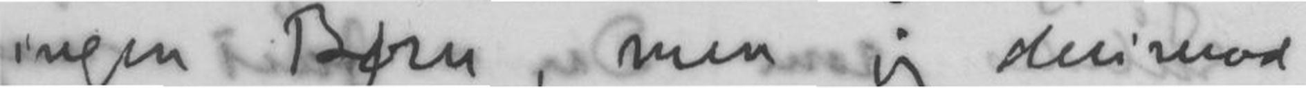ingen Børn, men jeg derimod
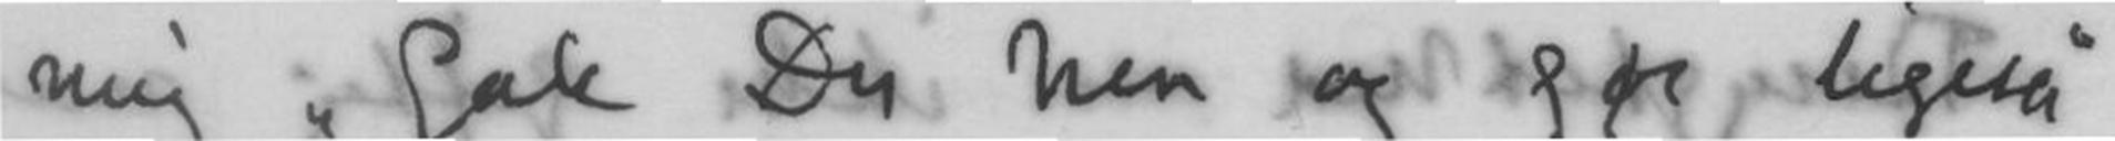mig Gak Du hen og gør ligeså
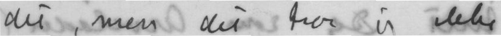det, men det tror vi ikke
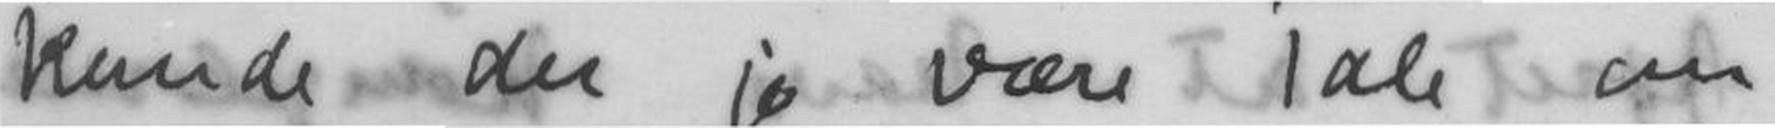kunde der jo være Tale om
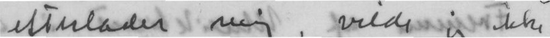efterlader mig, vilde jeg ikke
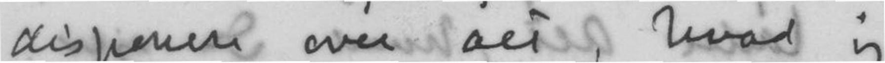disponere over alt, hvad jeg
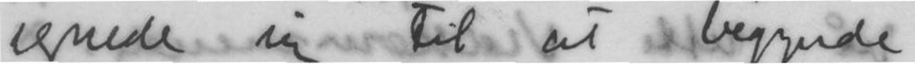egnede sig til at begynde
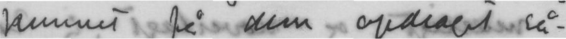kunnet få dem opdraget så-
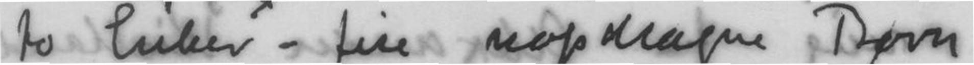to Enkerx - fire uopdragne Børn
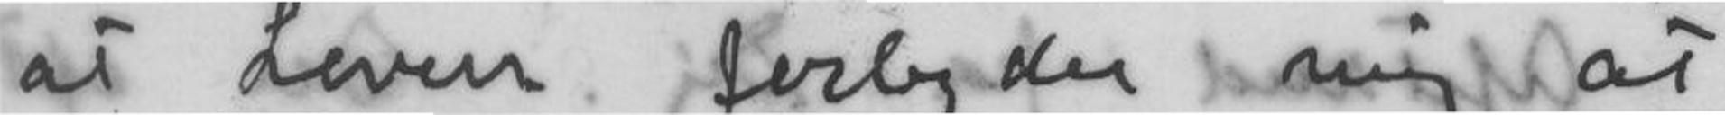at Loven forbyrder mig at
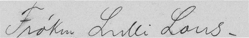Frøken Lulli Lous.
